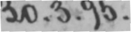30.3.95.
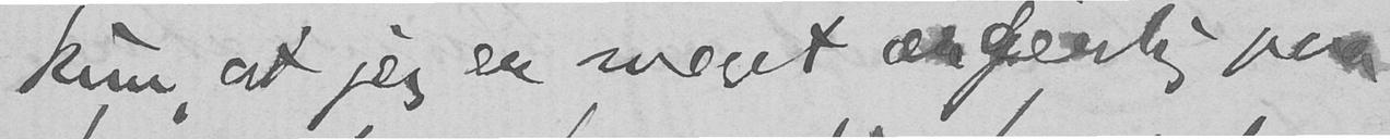kun, at jeg er meget ærgerlig paa
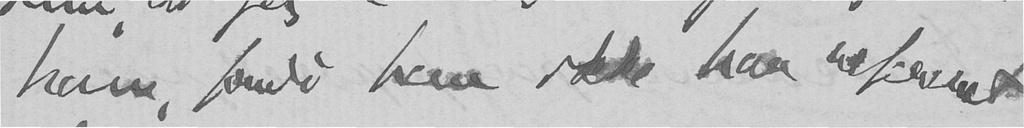ham, fordi han ikke har refereret
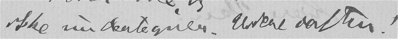ikke undertegner. Uskal daften!
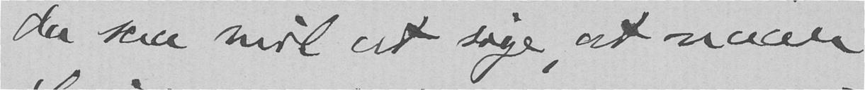da saa snil at sige, at naar
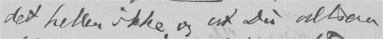det heller ikke, og at Du altsaa
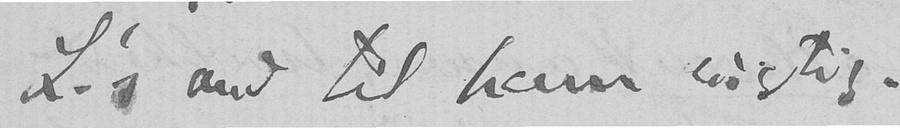L.'s ord til ham rigtig.
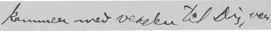kommer med vexelen til Dig, vær
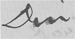Din
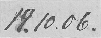19.10.06.
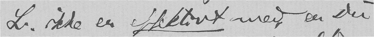L. ikke er effektivt med, er Du
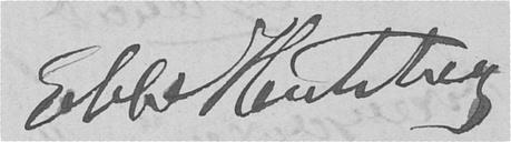Ebbe Hertzberg.
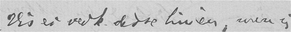Vis ei vedk. disse linier; men sig
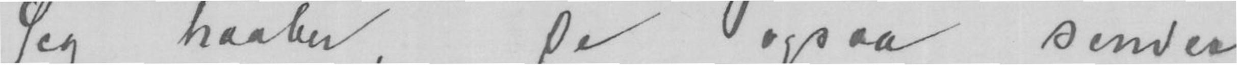jeg haaber, De ogsaa sender
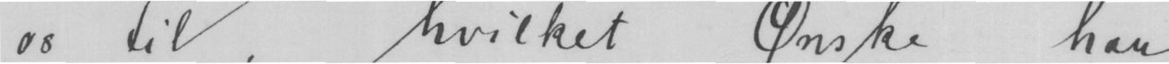os til, hvilket Ønske han
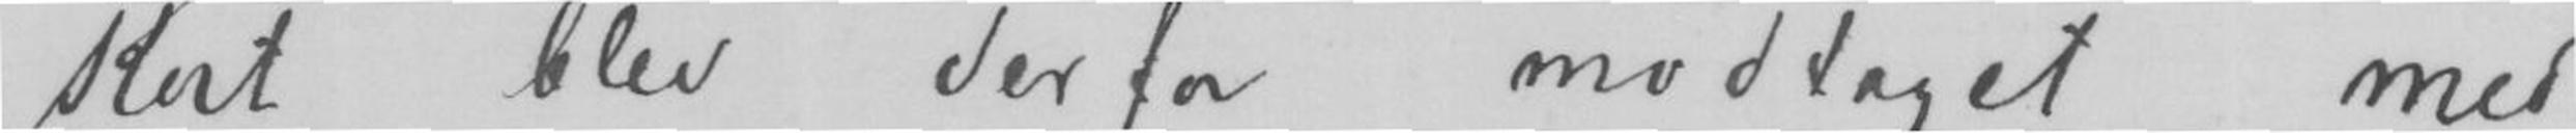Kort blev derfor modtaget med
